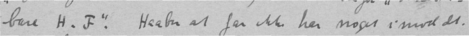bare H.F." Haaber at far ikke har noget imod det.
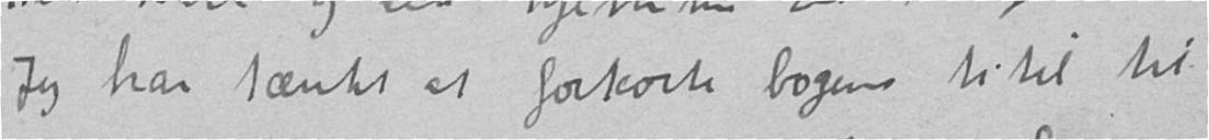Jeg har tænkt at forkorte bogens titel til
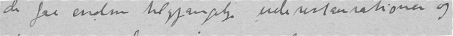de faa endnu tilgjængelige uderestaurationer og
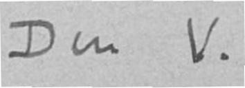Din V.
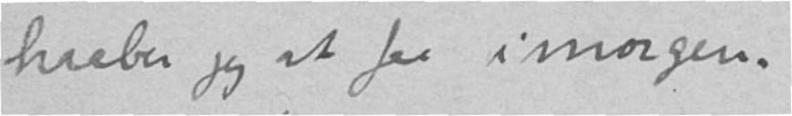haaber jeg at faa i morgen.
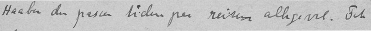Haaber du passer tiden paa reisen alligevel. Fik
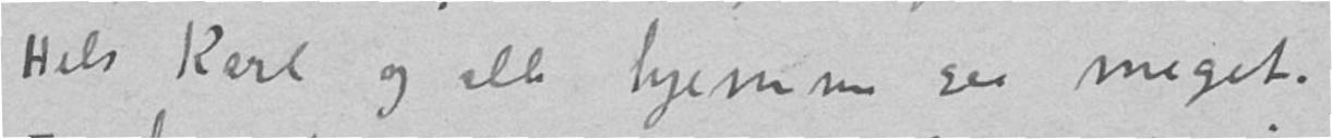Hils Karl og alle hjemme saa meget.
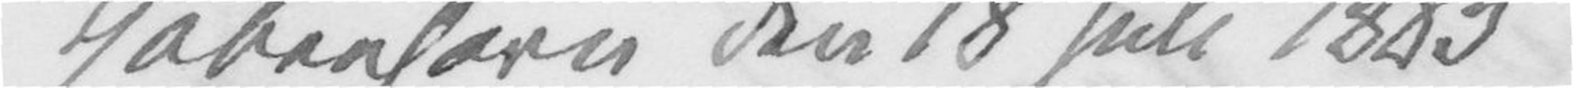Kjøbenhavn den 18 Juli 1883
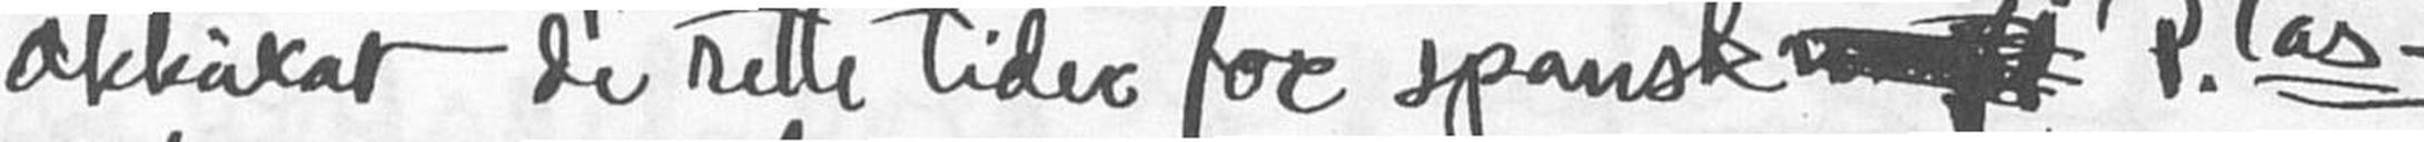akkurat de rette tider for spansk  P.tas -,
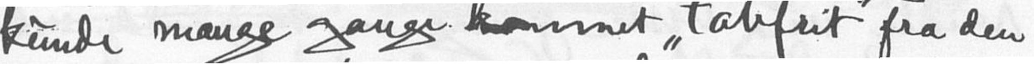kunde mange gange kommet "tabfrit" fra den
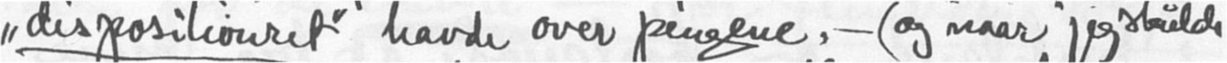"dispositionret" havde over pengene, - (og naar jeg skulde
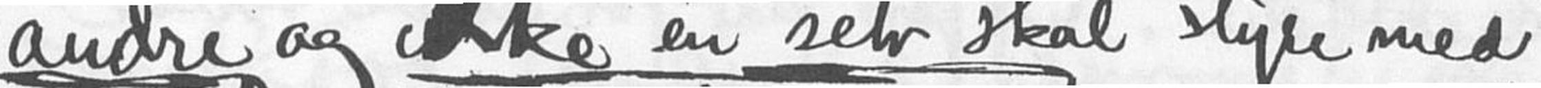andre og ikke en selv skal styre med
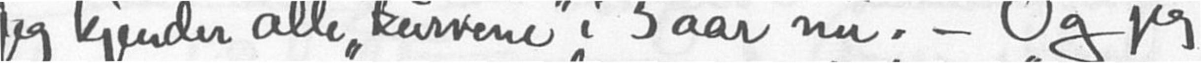jeg kjender alle "kursene" i 5 aar nu. - Og jeg
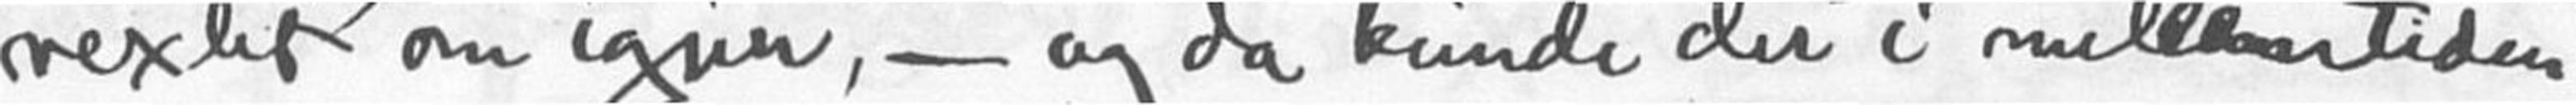vexlet om igjen, - og da kunde der i mellemtiden
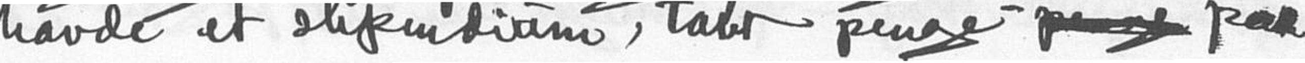havde et stipendium, tabt penge paa
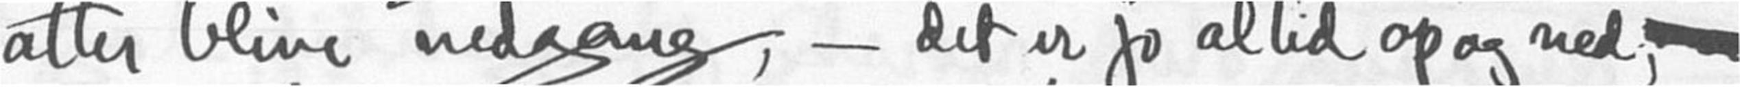atter blive nedgang, - det er jo altid op og ned, -
